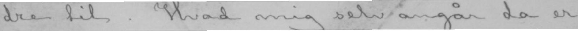dre til. Hvad mig selv angår da er
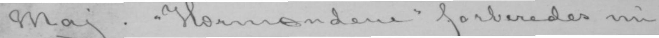i Maj. «Hærmændene» forberedes nu
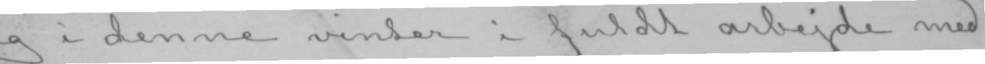jeg i denne vinter i fuldt arbejde med
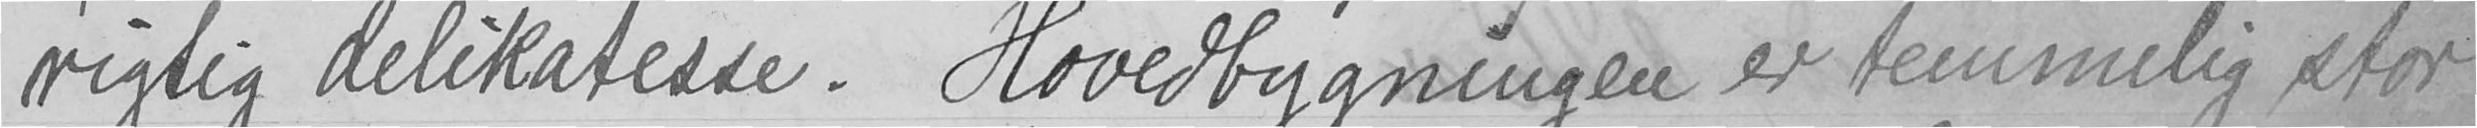rigtig delikatesse. Hovedbygningen er temmelig stor
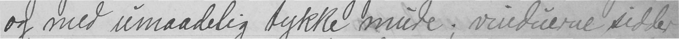og med umaadelig tykke mure, vinduerne sidder
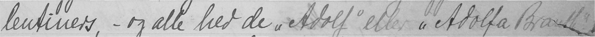lentiners, - og alle hed de "Adolf" eller "Adolfa Branth"
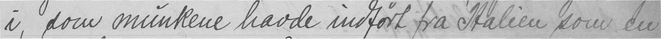i, som munkene havde indført fra Italien som en
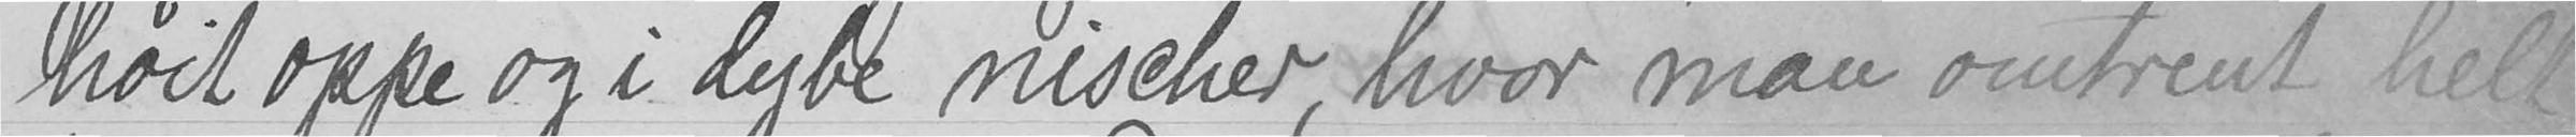høit oppe og i dybe nischer, hvor man omtrent helt
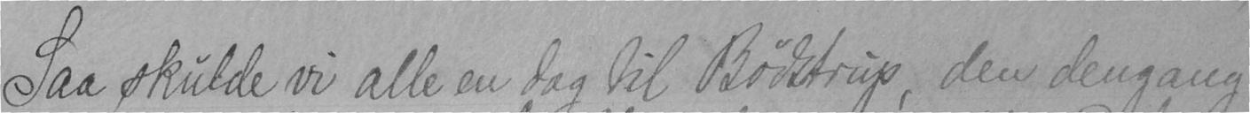Saa skulde vi alle en dag til Bødstrup, den dengang
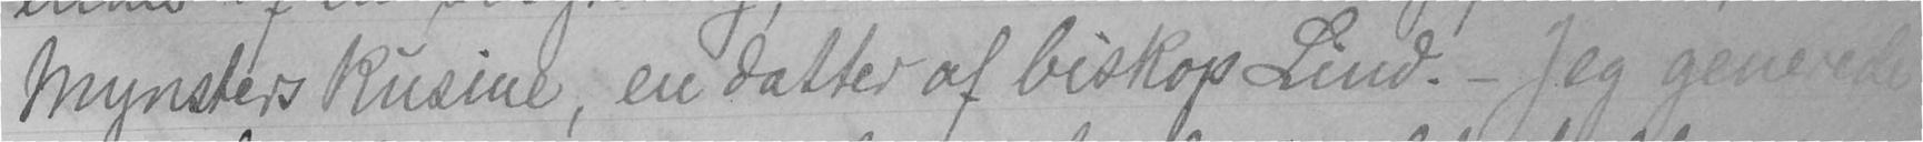Mynsters kusine, en datter af biskop Lund. - Jeg generede
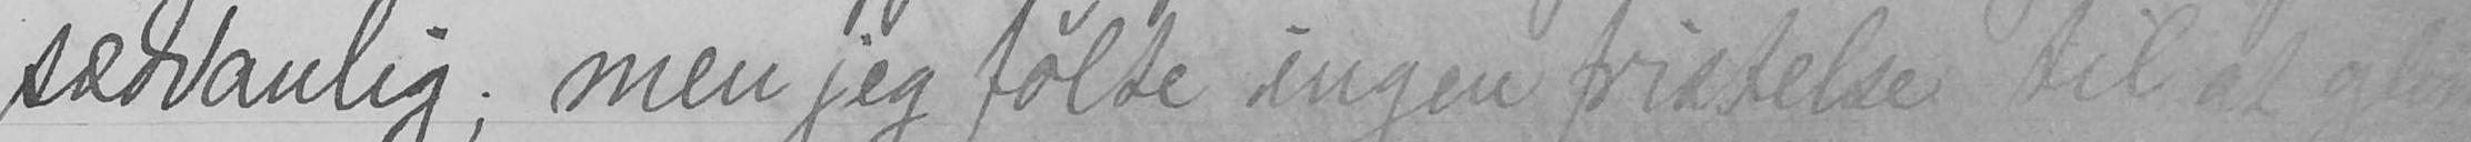sædvanlig; men jeg følte ingen fristelse til at glem
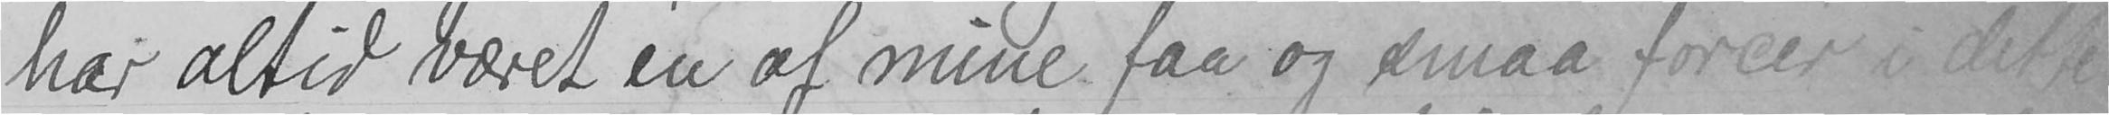har altid været en af mine faa og smaa forcer i dette
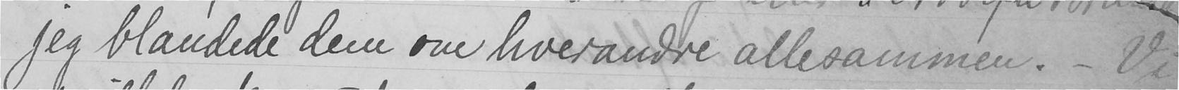jeg blandede dem om hverandre allesammen. - Vi
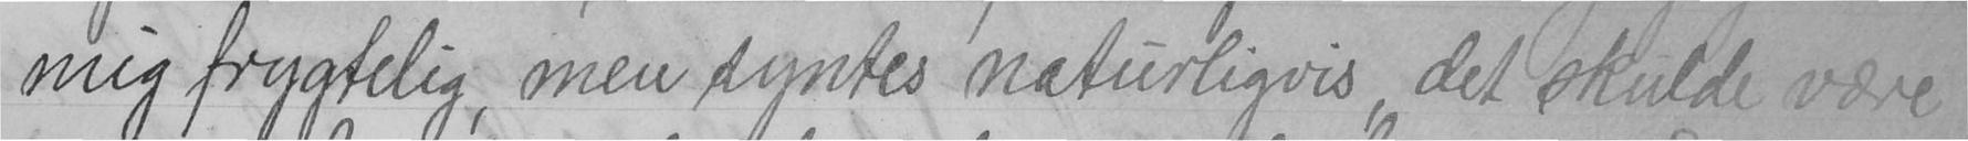mig frygtelig, men syntes naturligvis det skulde være
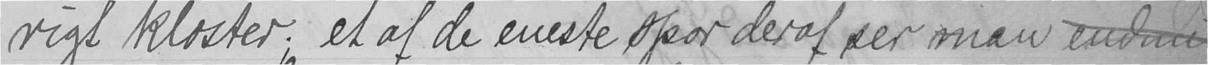rigt kloster; et af de eneste spor deraf ser man endnu
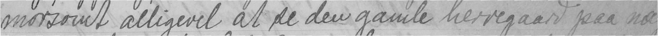morsomt alligevel at se den gamle herregaard paa næ
